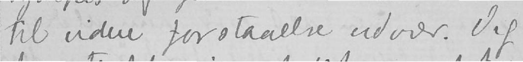til videre forstaaelse udover. Jeg
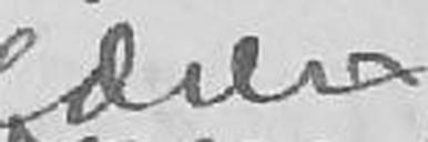læren
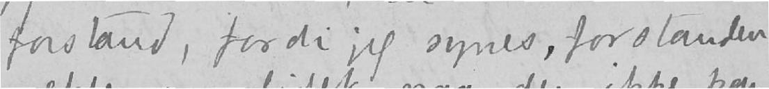forstand, fordi jeg synes, forstanden
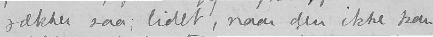rækker saa lidet, naar den ikke kan
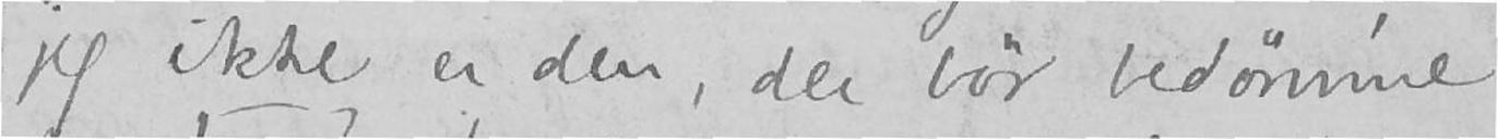jeg ikke er den, der bør bedømme
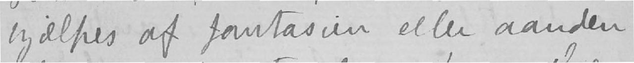hjælpes af fantasien eller aanden
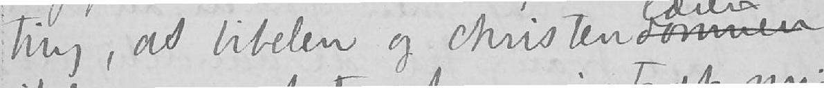ting, at bibelen og christendommen
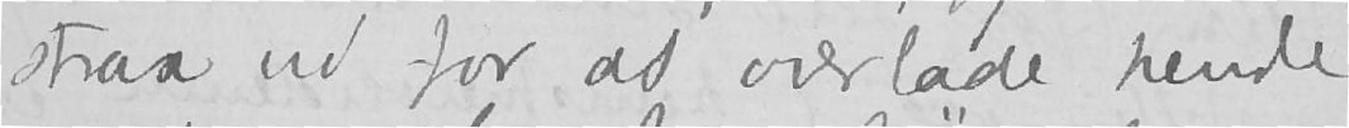strax ud for at overlade hende
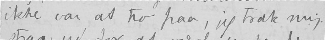ikke var at tro paa, jeg trak mig
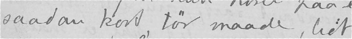saadan kort, tør maade, lidt
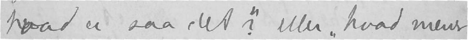«hvad er saa det?» eller «hvad mener
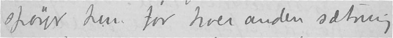spørger hun for hver anden sætning
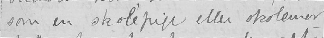som en skolepige eller skolemor
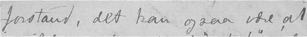forstand, det kan ogsaa være, at
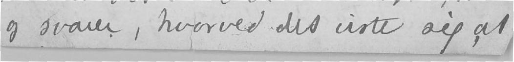og svarer, hvorved det viste sig, at
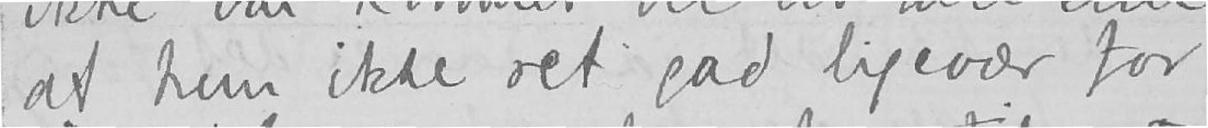at hun ikke ret gad ligeover for
